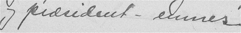og præsident-emnet
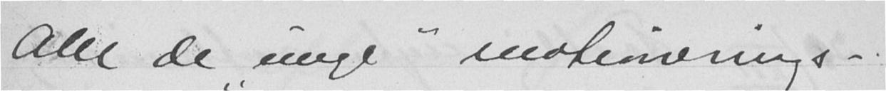Alle de "unge" motionerings-
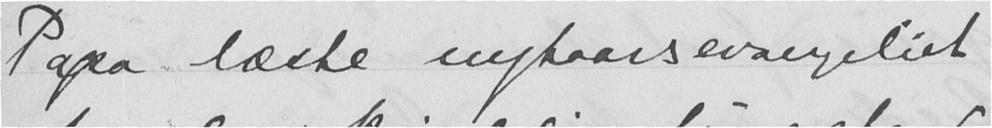Papa læste nytaarsevangeliet
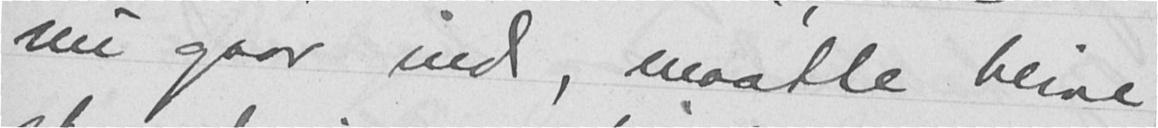nu gaar ind, maatte blive
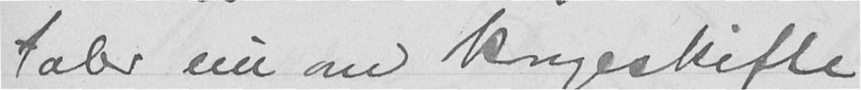taler nu om kongeskifte
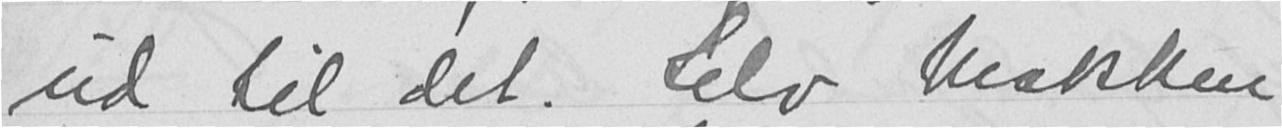ud til det. Selv Makken
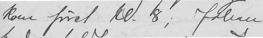kom først kl. 8; Johan
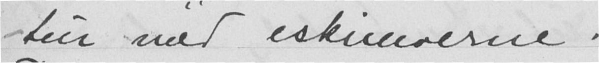tur med eskimoerne.
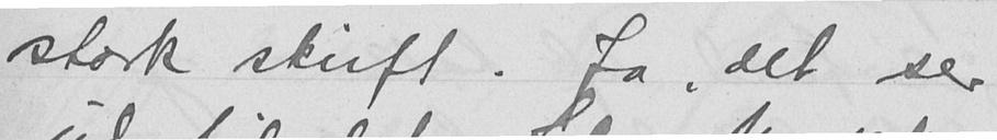stork skrift. Ja, det ser
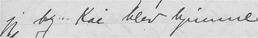jeg og Kai blev hjemme
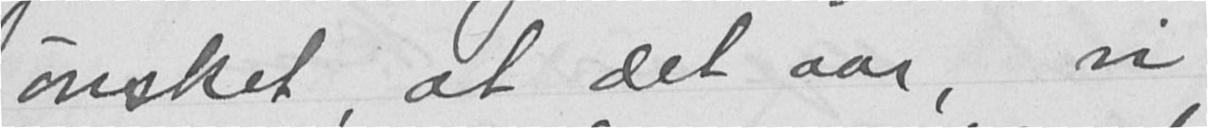ønsket at det aar, vi
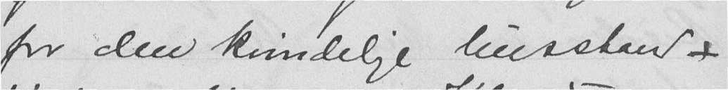for den kvindelige husstand -
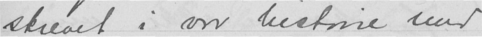skrevet i vor historie med
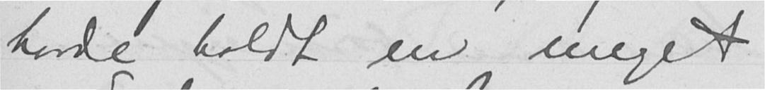havde holdt en meget
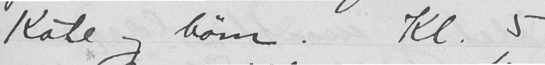Kate og børn. Kl. 5
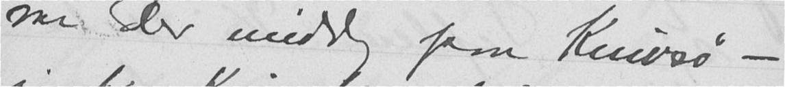var der middag paa Knivsø -
